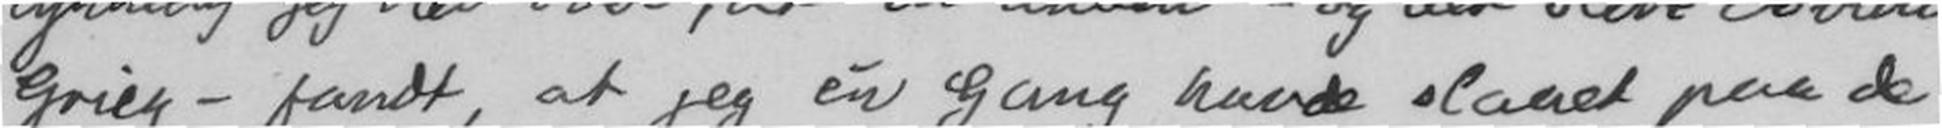Grieg - fandt, at jeg én Gang kunde slaaet paa de
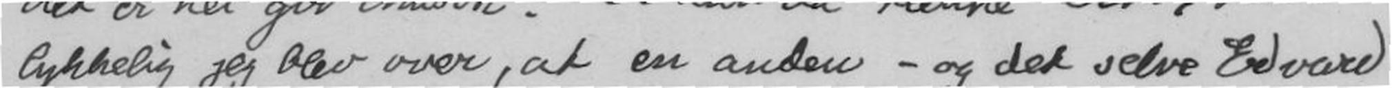lykkelig jeg blev over, at en anden - og det selve Edvard
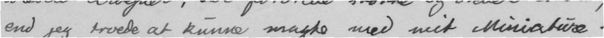en jeg troede at kunne magte med mit Miniature -
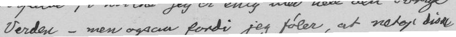Verden - men ogsaa fordi jeg føler, at netop disse
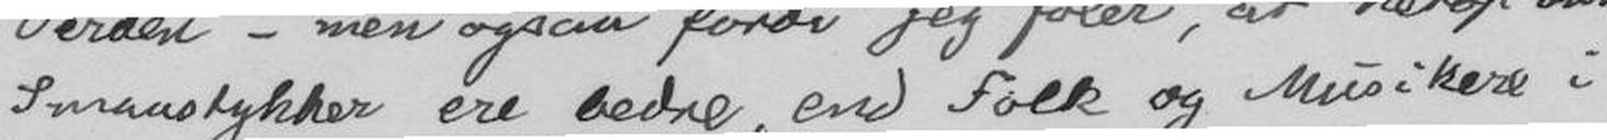Smaastykker ere bedre, end Folk og Musikere i
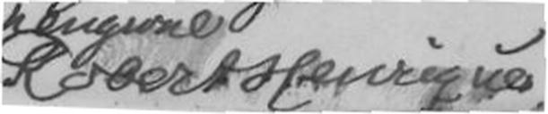Robert Henriques
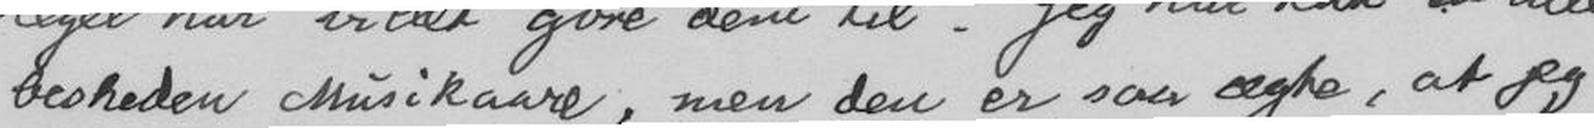beskeden Musikaare, men den er saa ægte, at jeg
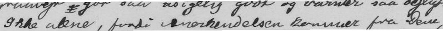Ikke alene, fordi amerkendelsen kommer fra Dem,
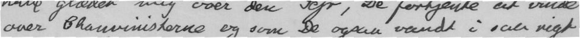over Ghauvinisterne og som De ogsaa vandt i saa rigt
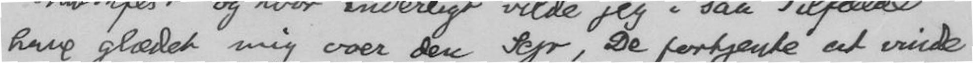have glædet mig over den Sejr, De fortjente at vinde
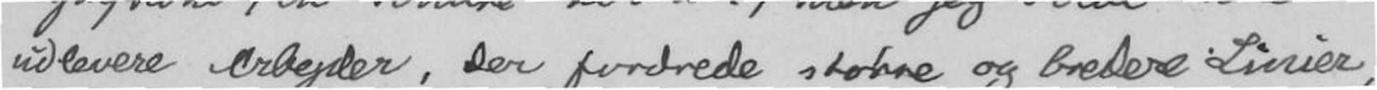udlevere arbejder, der fordrede større og bredere Linier,
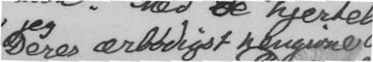Deres ærbødigst hengivne
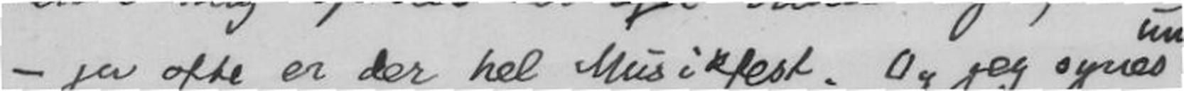- ja ofte er der hel Musikfest. Og jeg synes
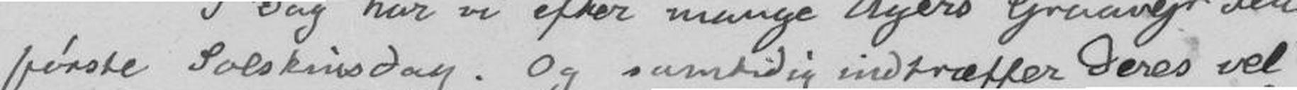første Solskinsdag. Og samtidig indtræffer Deres vel-
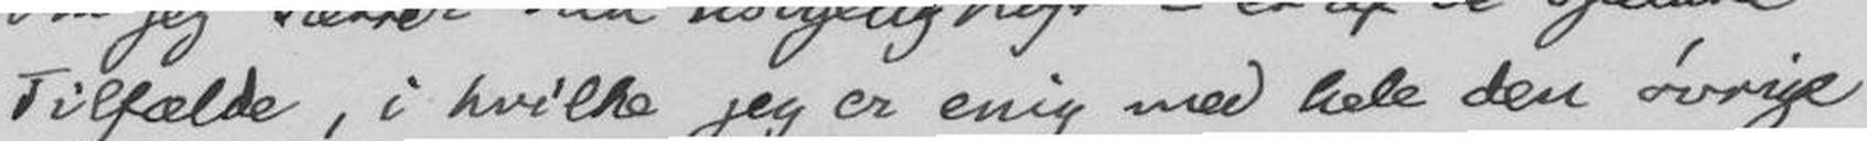Tilfælde, i hvilke jeg er enig med hele den øvrige
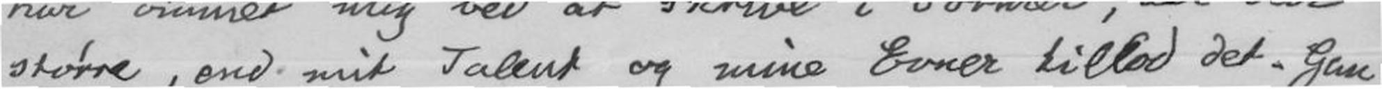større, end mit talent og mine Evner tillod det. Gan-
